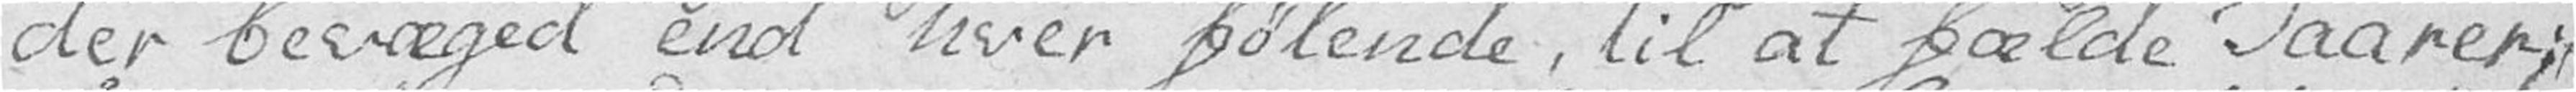der bevæged end hver følende, til at fælde Taarer;
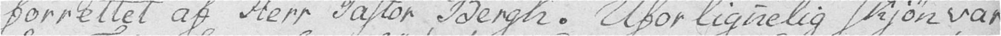forrettet af Herr Pastor Bergh. Uforlignelig skjøn var
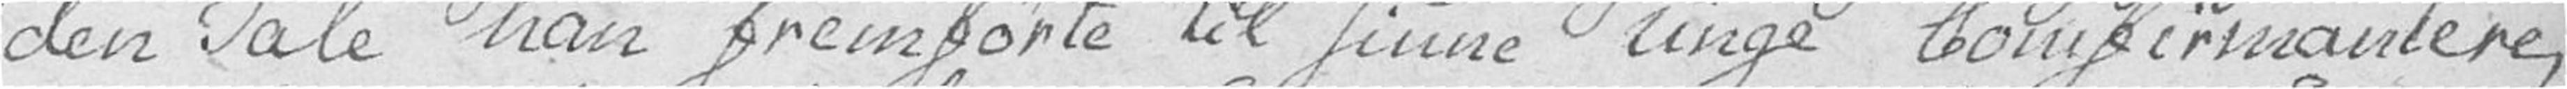den Tale han fremførte til sinne unge Comfirmantere,
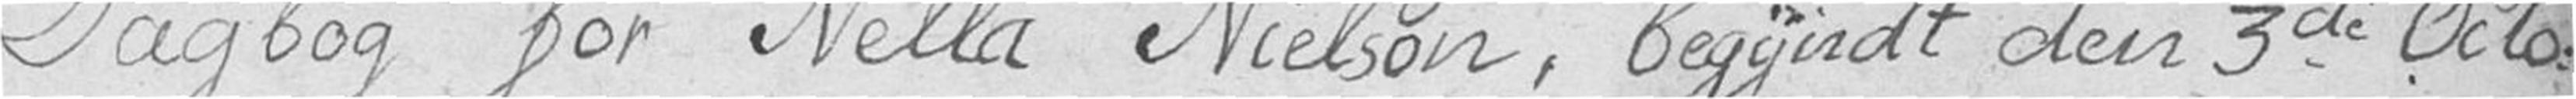Dagbog for Nella Nielson, begyndt den 3di Octo.
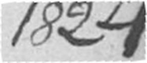1824
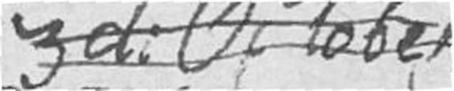3di October
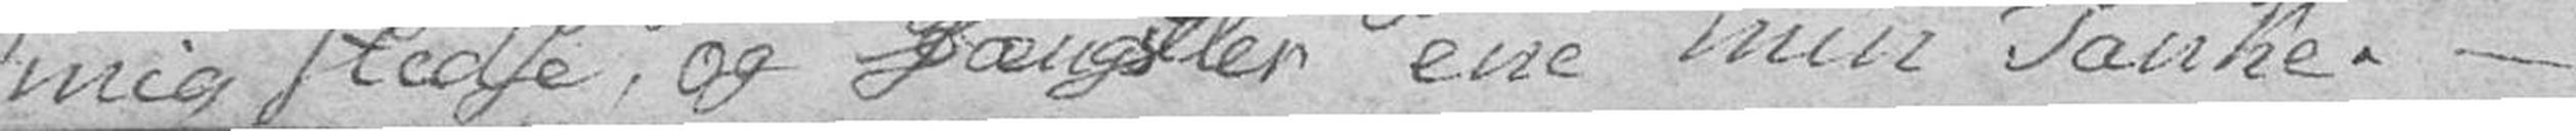mig stedse, og fængsler ene min Tanke. –
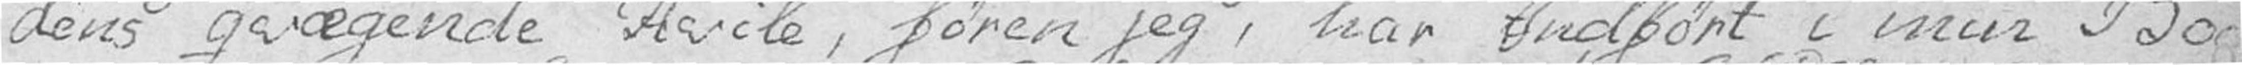dens qvægende Hvile, føren jeg, har Indført i min Bog
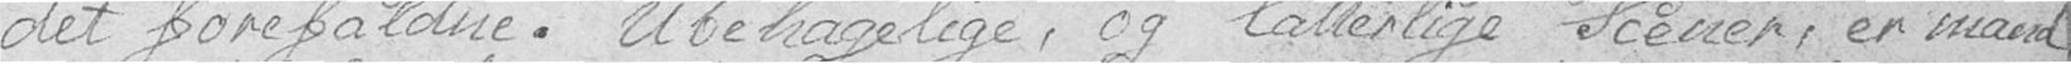det forefaldne. Ubehagelige, og latterlige Scener, er mand
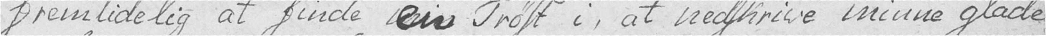fremtidelig at finde en Trøst i, at nedskrive minne glade
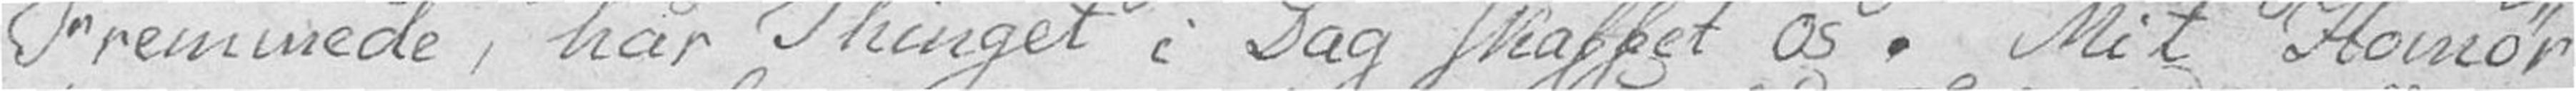Fremmede, har Thinget i Dag skaffet os. Mit Homør
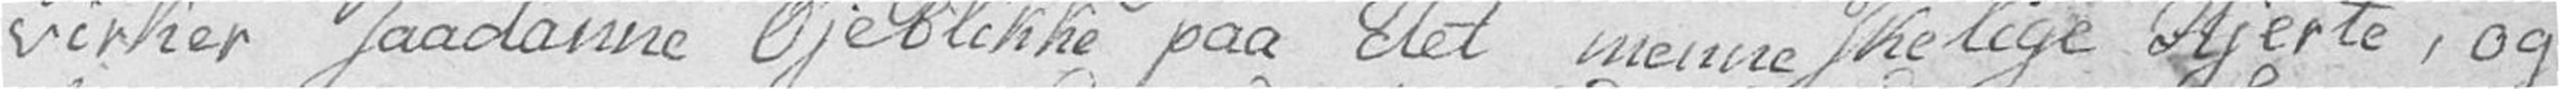virker saadanne Øjeblikke paa det menneskelige Hjerte, og
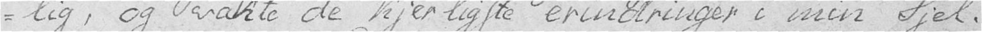-lig, og vakte de kjerligste erindringer i min Sjel. –
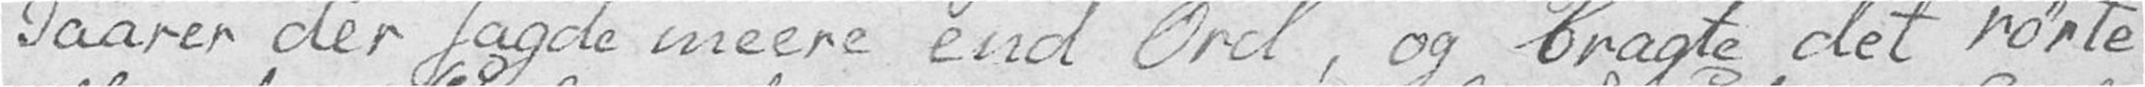Taarer der sagde meere end Ord, og bragte det rørte
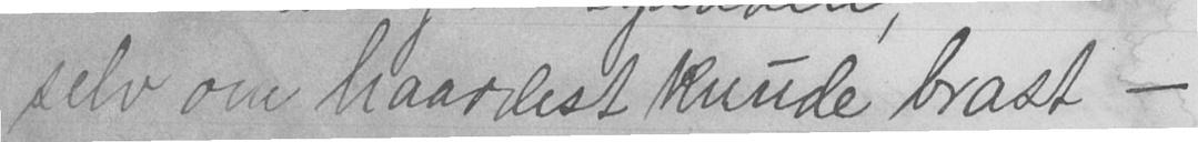selv om haardest knude brast -
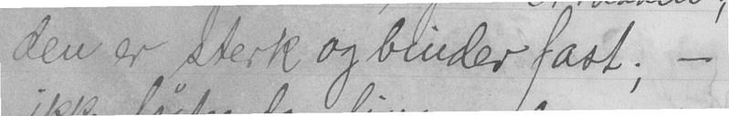den er sterk og binder fast; -
